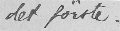det første.
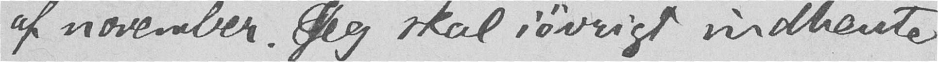af november. Jeg skal iøvrigt indhente
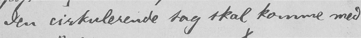Den cirkulerende sag skal komme med
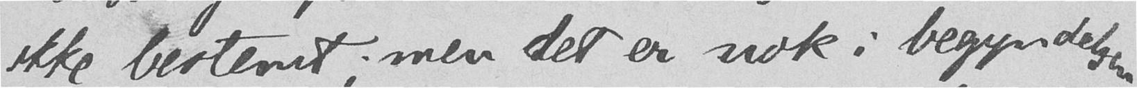ikke bestemt; men det er nok i begyndelsen
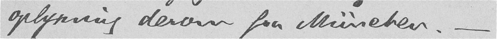oplysning derom fra München. -
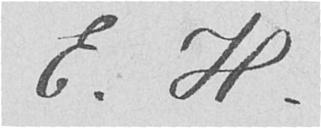E. H.
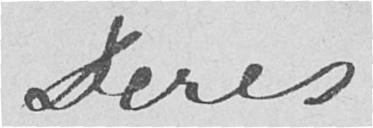Deres
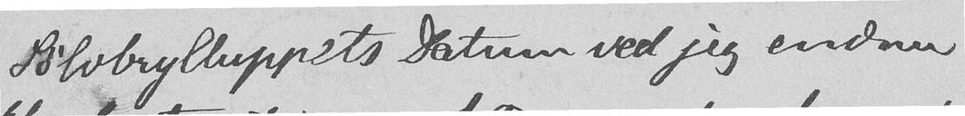Sølvbrylluppets Datum ved jeg endnu
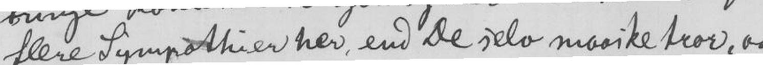flere Sympathier her, end De selv maaske tror, og
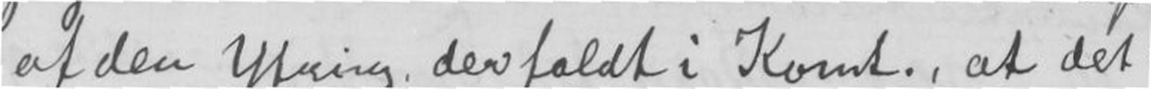af den Ytring der faldt i Komt., at det
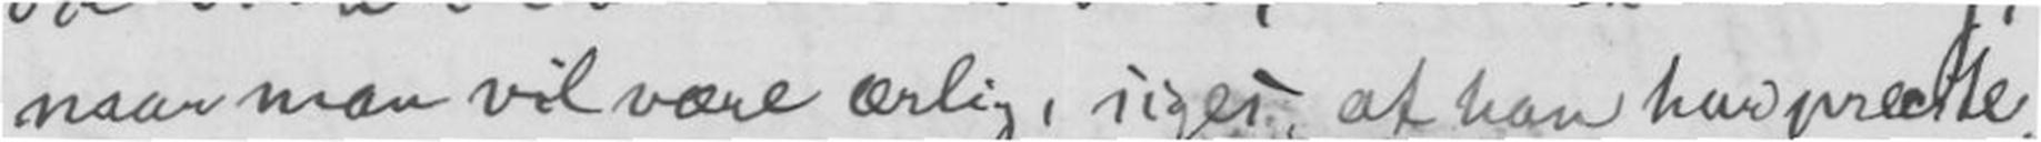naar man vil være ærlig, siges at han har præste-
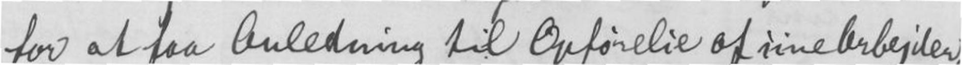for at faa Anledning til Opførelsen af sine Arbejder
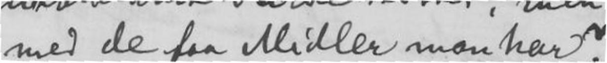med de faa midler man har?
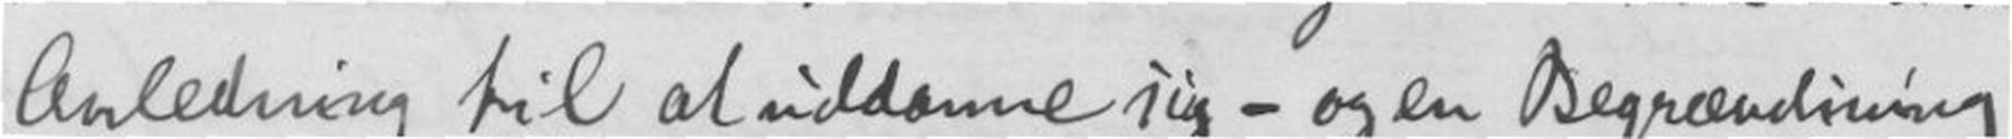Anledning til at uddanne sig - og en Begrændsning
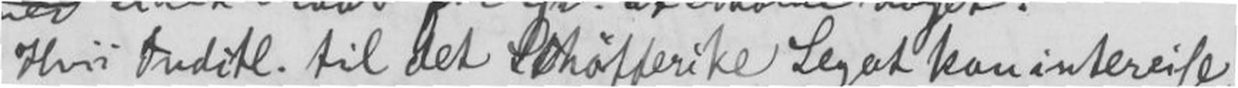Hvis Indstl. til det Schøfferske Legat kan interesse-
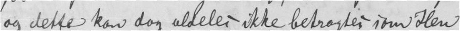og dette kan dog aldeles ikke betragtes som Hen-
